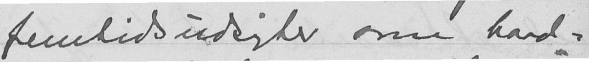fremtidsudsigter som baad-
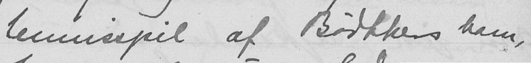tennisspil af Bødtkers barn,
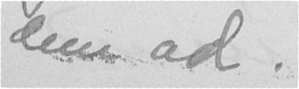dem ad.
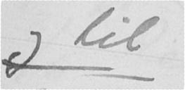og til
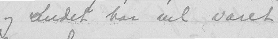og andet har vel varet
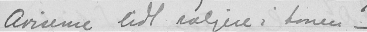Aviserne lidt roligere i tonen -
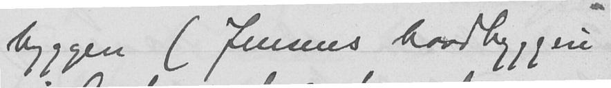bygger (Jensens baadbyggeri
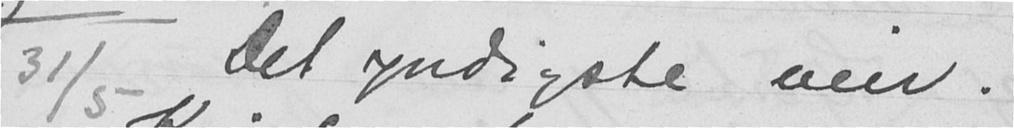31/5 Det yndigste veir.
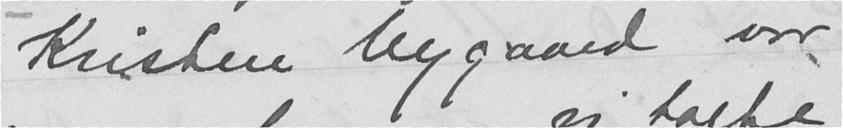Kristen Nygaard var
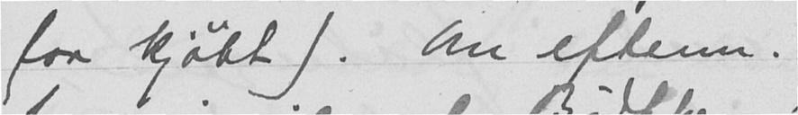faa kjøbt). Om efterm.
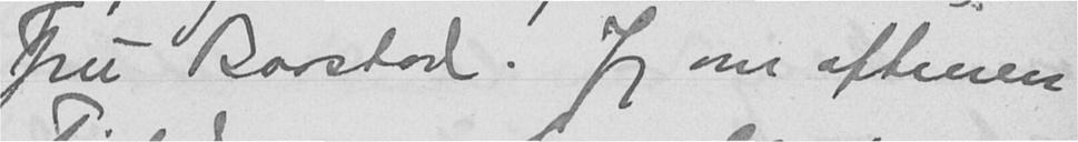Fru Baastad. Jeg om aftenen
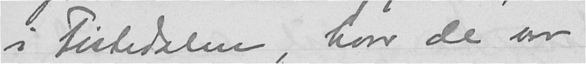i Tistedalen, hvor de var
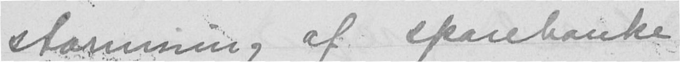stormning af sparebanke
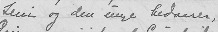Leni og den unge bedaarer,
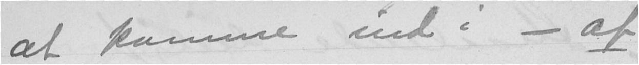at komme ind i - af
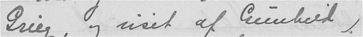Grieg, og visit af Gunhild,
